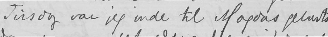Tirsdag var jeg inde til Magdas geburts-
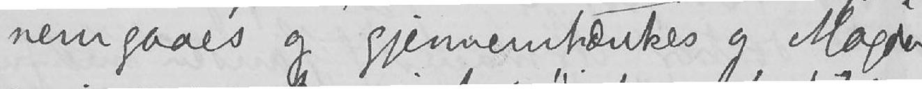nemgaaes og gjennemtænkes og Magda
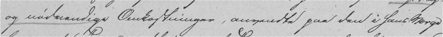og nødvendige Omkostninger, anvendte paa den i hans Værge
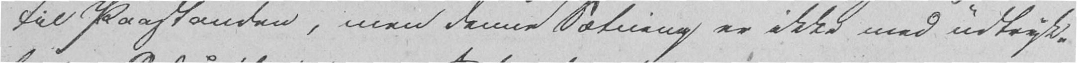til Paastanden, men denne Sætning er ikke med udtryk-
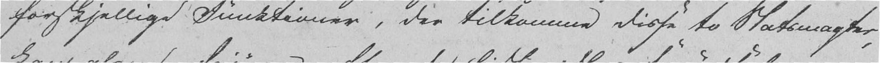forskjellige Funktioner, der tilkomme disse to Statsmagter,
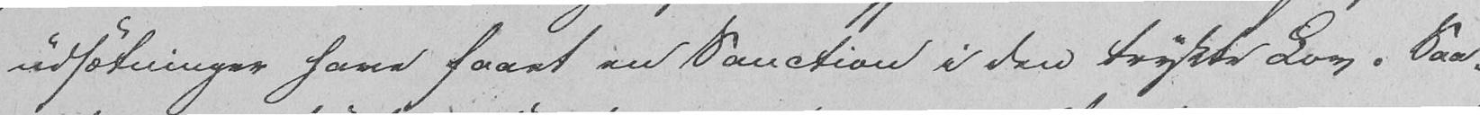udsætninger have faaet en Sanction i den trykte Lov. Saa-
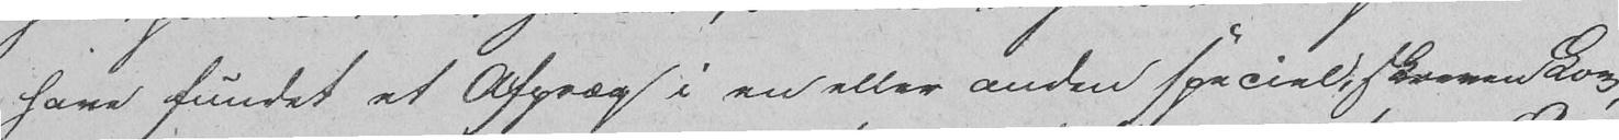have fundet et Afpræg i en eller anden speciel, skreven Lov;
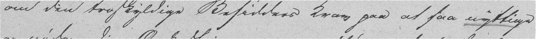om den troskyldige Besidders krav paa at faa nyttige
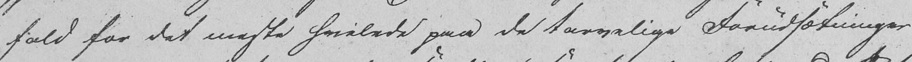fald for det meste hvilede paa de tarvelige Forudsætninger
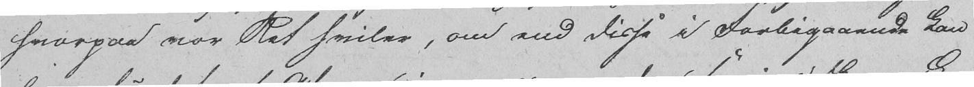hvorpaa vor Ret hviler, om end disse i Forbigaaende kan
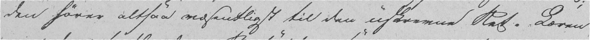den hører altsaa væsentligst til den uskrevne Ret. Loven
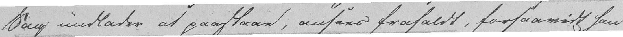Sag undlader at paastaae, ansees frafaldt, forsaavidt han
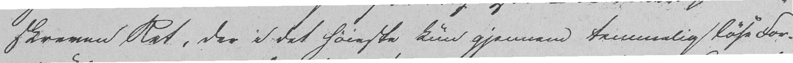skreven Ret, der i det høieste kun gjennem temmelig løse For-
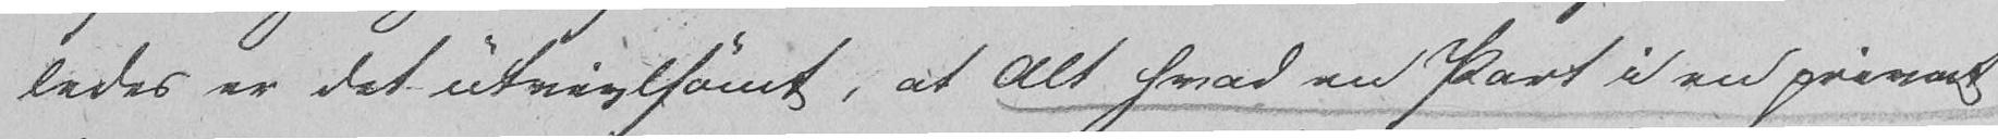ledes er det utvivlsomt, at alt hvad en Part i en privat
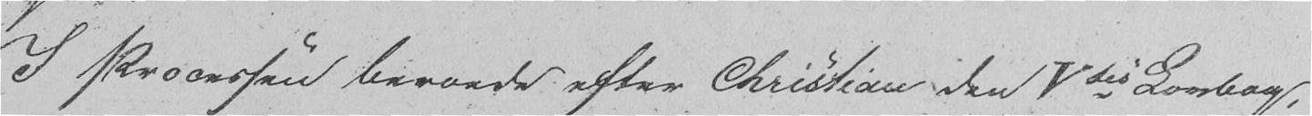I Processen beroede efter Christian den Vdes Lovbog,
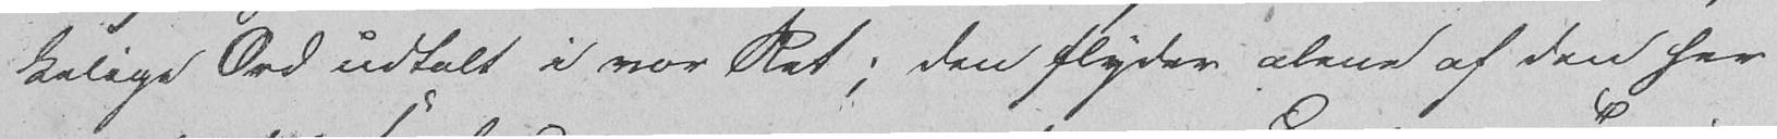kelige Ord udtalt i vor Ret; den flyder alene af den her
